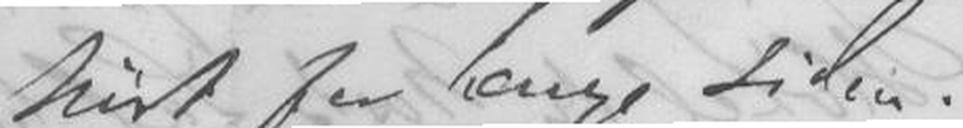hørt for længe siden.
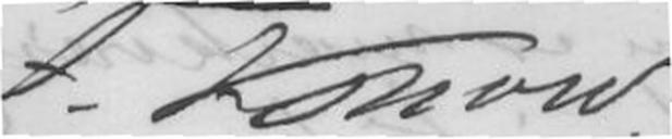F. Konow.
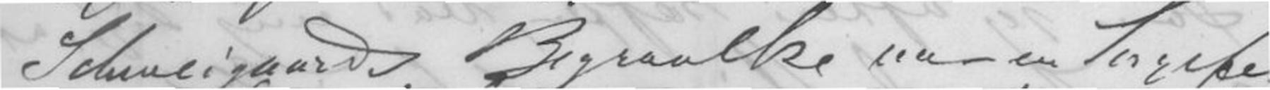Schweigaard Begravelse var en Sorgefe
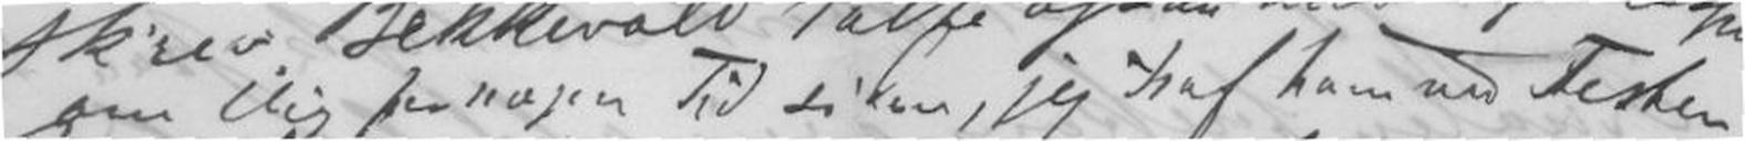om Dig for nogen Tid siden, jeg traf hem ved Festen.
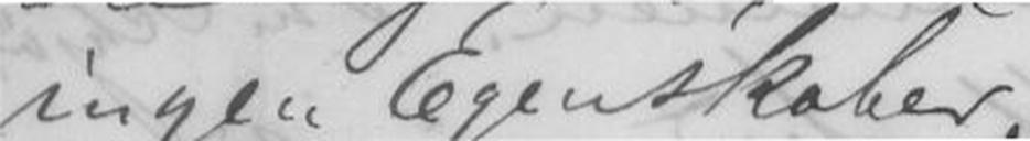ingen Egenskaber.
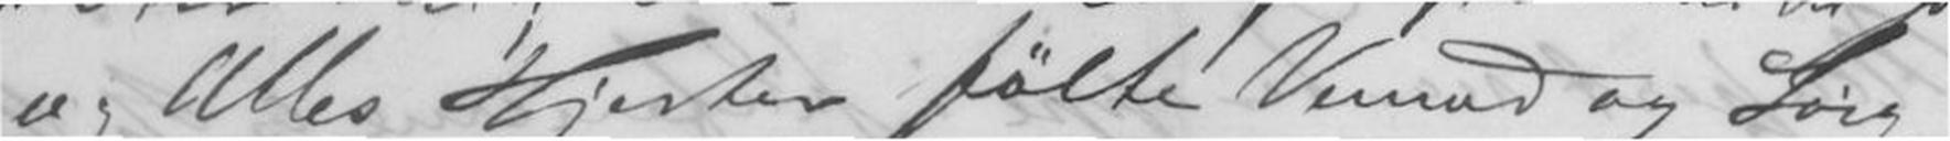og Alles Hjerter følte Vemod og Sorg
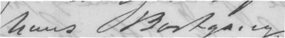hans Bortgang.
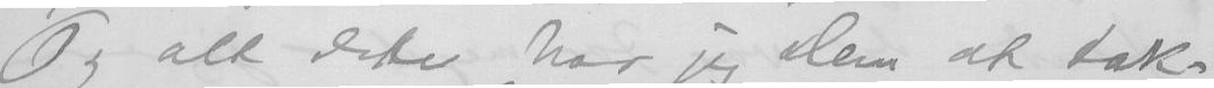Og alt dette har jeg Dem at tak-
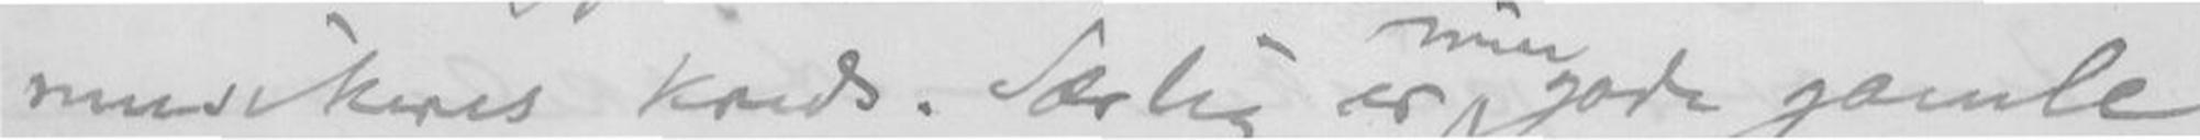musikeres kreds. Særlig er gode gamle
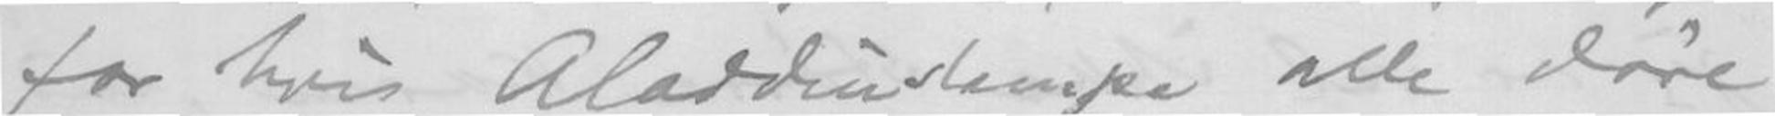for hvis Alladdinslampe alle døre
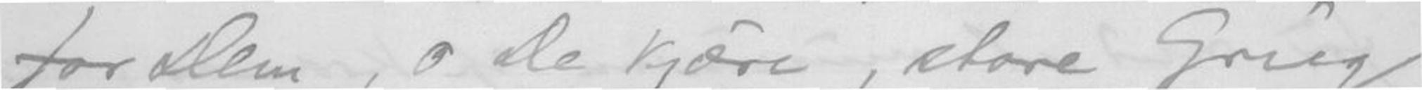for Dem, o De kjære, store Grieg,
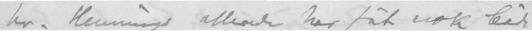hr. Hennings allerede har fåt nok både
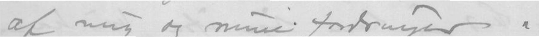af mig og mine fordringer.
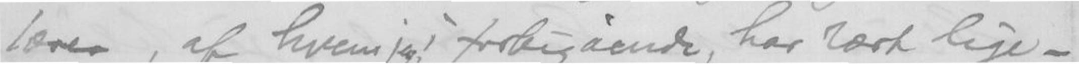lærer, at hvem jeg forbigående, har læst lige-
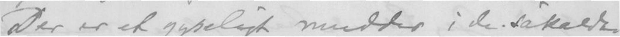Der er et gyseligt mudder i de såkaldtes
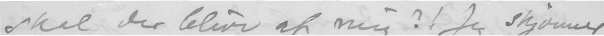skal der blive af mig?! Jeg skjønner
